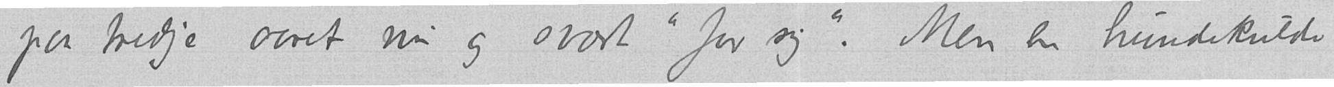paa tredje aaret nu og svært "for sig". Men en hundekulde
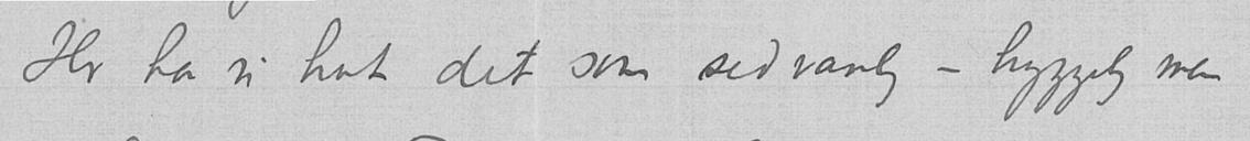Her har vi hat det som sedvanlig – hyggelig men
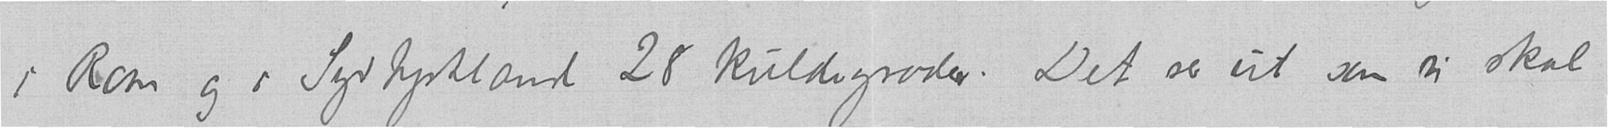i Rom og i Sydtyskland 28 kuldegrader. Det ser ut som vi skal
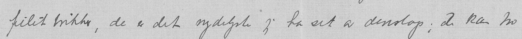filetbrikker, de er det nydeligste jeg har set av denslags; de kan tro
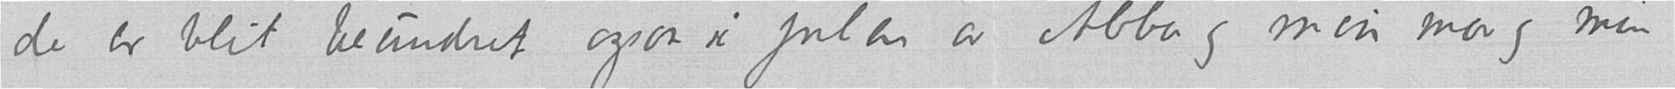de er blit beundret ogsaa i julen av Abba og min mor og min
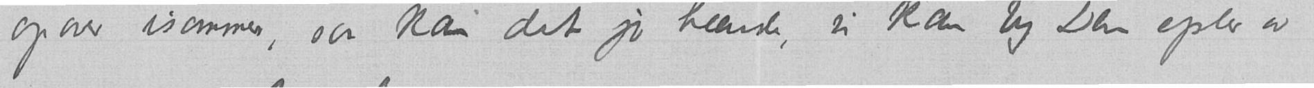opover isommer, saa kan det jo hende, vi kan by Dem epler av
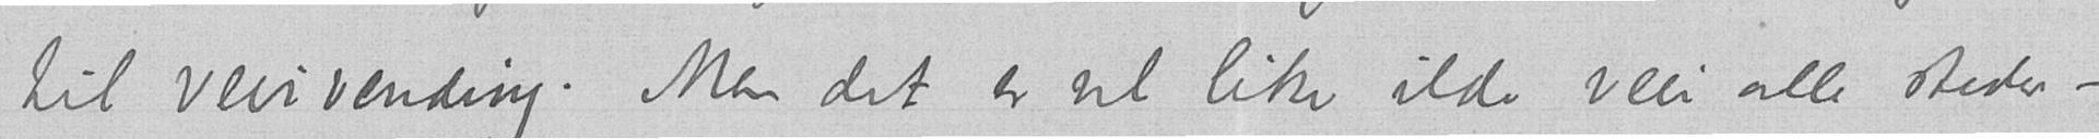til veirvending. Men det er vel like ilde veir alle steder –
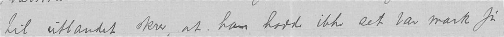til utlandet skrev, at han hadde ikke set bar mark før
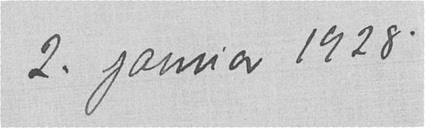2. januar 1928.
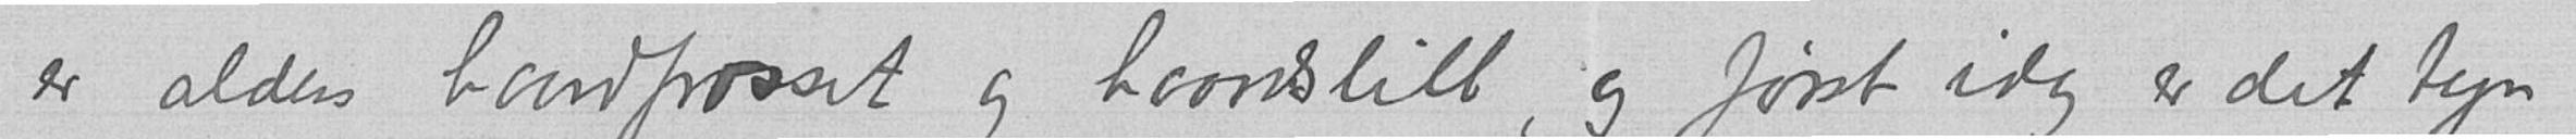er aldeles haardfrosset og haardslitt, og først idag er det tegn
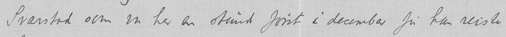Svarstad som var her en stund først i december før han reiste
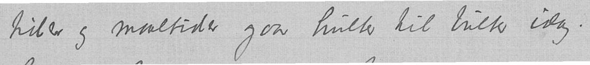tider og maaltider gaar hulter til bulter idag.
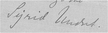Sigrid Undset.
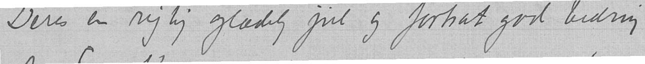Deres en rigtig glædelig jul og fortsat god bedring
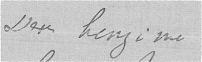Deres hengivne
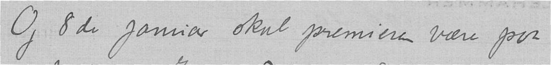Og 8de januar skal premieren være paa
